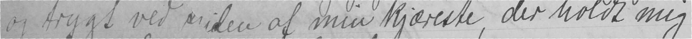og trygt ved siden af min kjæreste, der holdt mig
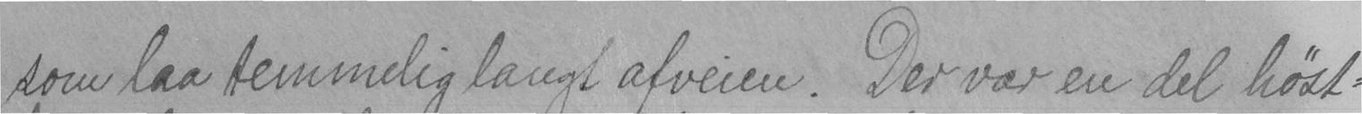som laa temmelig langt afveien. Der var en del høst-
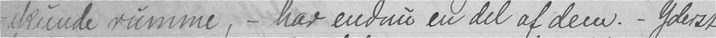kunde rumme, - har endnu en del af dem. - Yderst
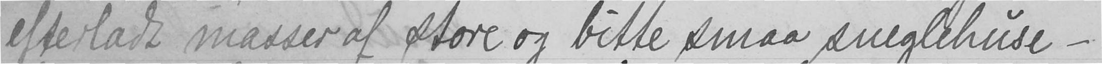efterladt masser af store og bitte smaa sneglehuse -
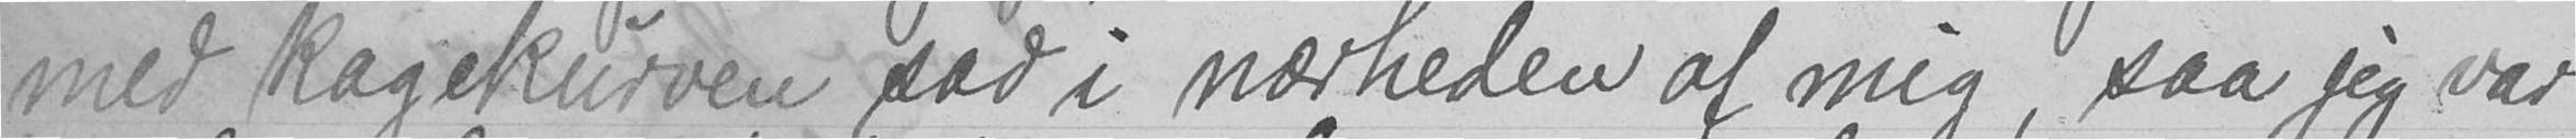med kagekurven sad i nærheden af mig, saa jeg var
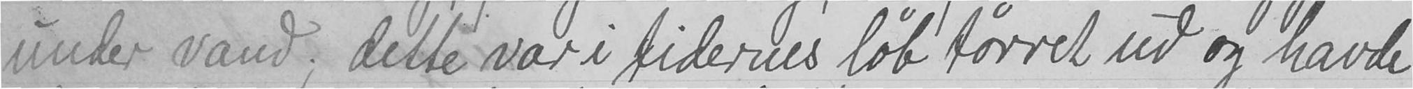under vand; dette var i tidernes løb tørret ud og havde
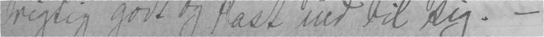riktig godt og fast ind til sig. -
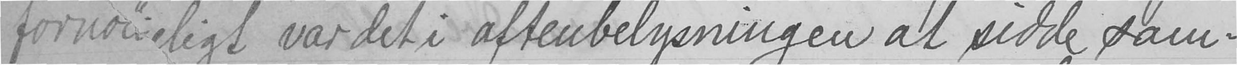fornøieligt var det i aftenbelysningen at sidde sam-
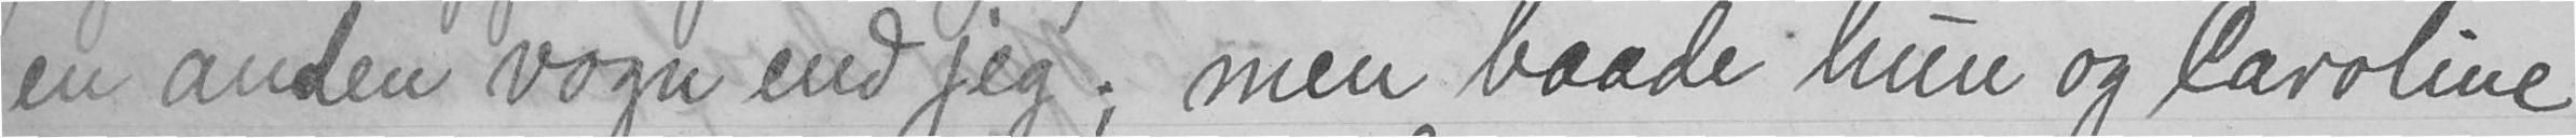en anden vogn end jeg; men baade hun og Caroline
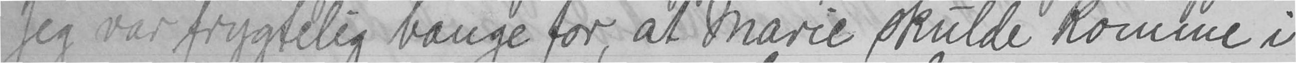Jeg var frygtelig bange for, at Marie skulde komme i
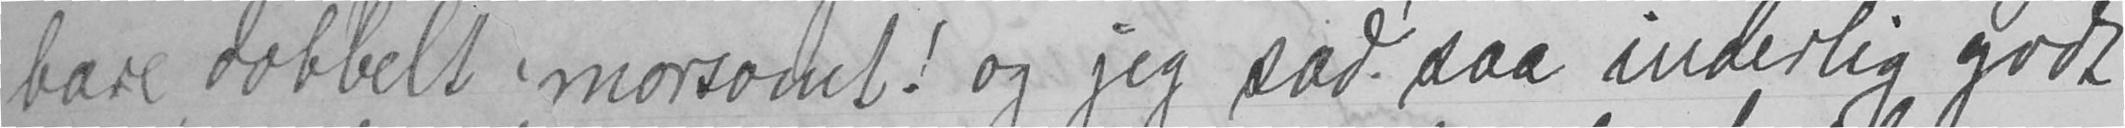bare dobbelt morsomt! og jeg sad saa inderlig godt
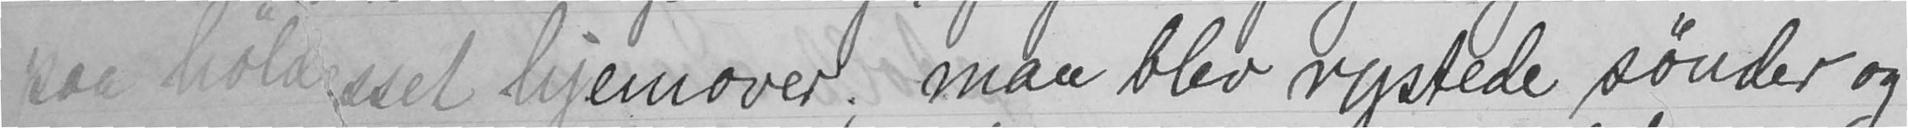paa hølæsset hjemover; man blev rystede sønder og
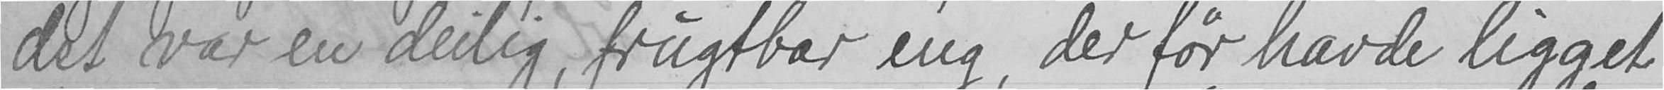det var en deilig, frugtbar eng, der før havde ligget
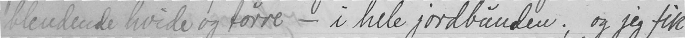blendende hvide og tørre - i hele jordbunden; og jeg fik
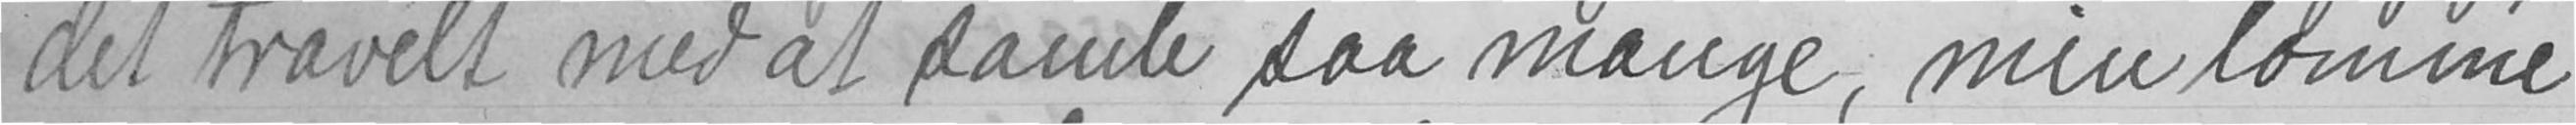det travelt med at samle saa mange, min lomme
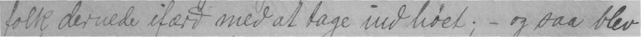folk dernede ifærd med at tage ind høet;- og saa blev
